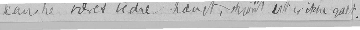kanske været bedre hængt, skjønt det er ikke galt.
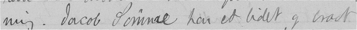ning. Jacob Sømme har et lidet og bravt
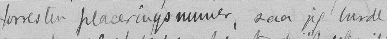forresten placeringsnumer, saa jeg burde
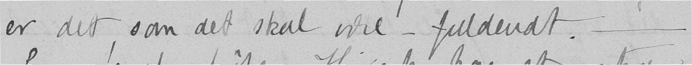er det, som det skal være - fuldendt. -
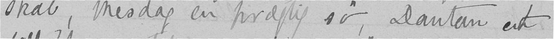skab, Mesdag en prægtig sø, Dantan et
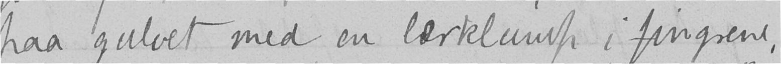paa gulvet med en lærklump i fingrene,
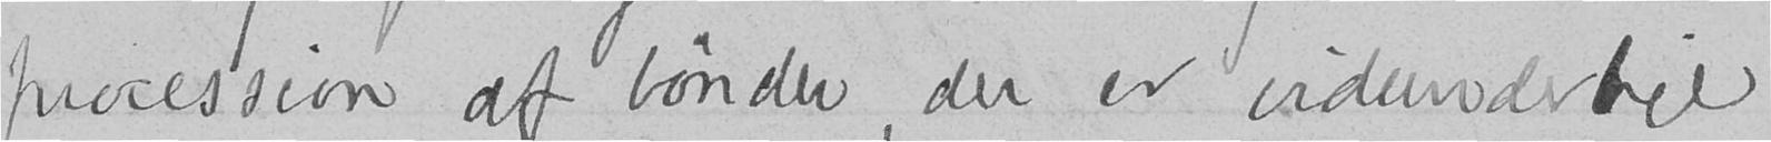procession af bønder, der er vidunderlie
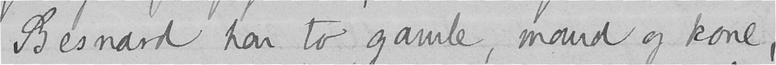Besnard har to gamle, mand og kone,
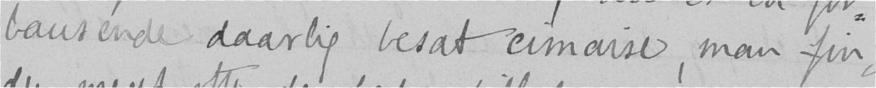bausende daarlig besat cimaise, man fin-
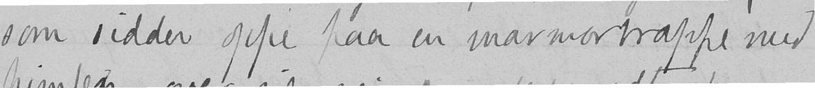som sidder oppe paa en marmortrappe med
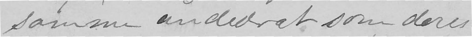samme åndedræt som deres
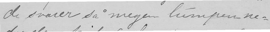de svarer så megen lumpen ne-
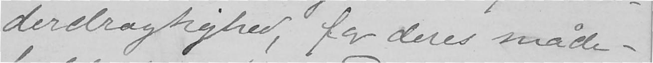derdrægtighed, for deres måde-
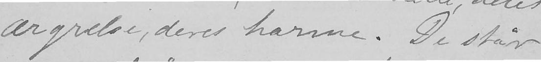ærgrelse, deres harme. De står
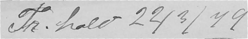Fr.hald 22/3/79
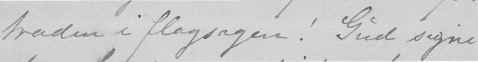træden i flagsagen! Gud signe
